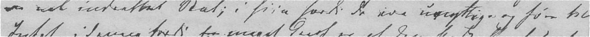en vel indrettet Stat; i hiin fordi de ere unyttige og føre til
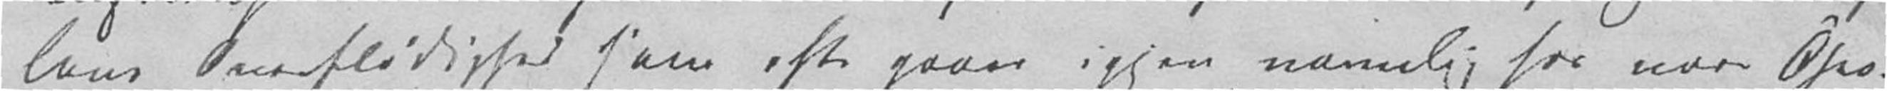Lovs Overflødighed som ofte gaaer igjen nemlig hos vore Theo-
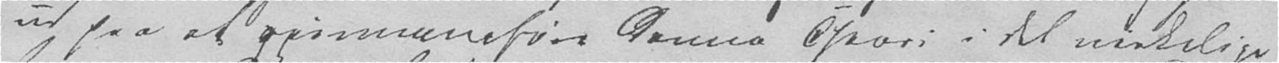ud paa at gjemføre denne Theori i det virkelige
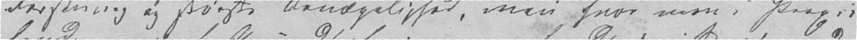Forskning og største Bevægelighed, men hvor man i Praxis
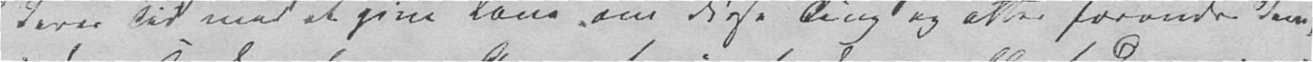deres Tid med at give Love om disse Ting og atter forandre dem,
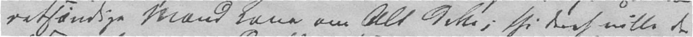retfærdige Mænd Love om Alt dette; thi deraf ville de
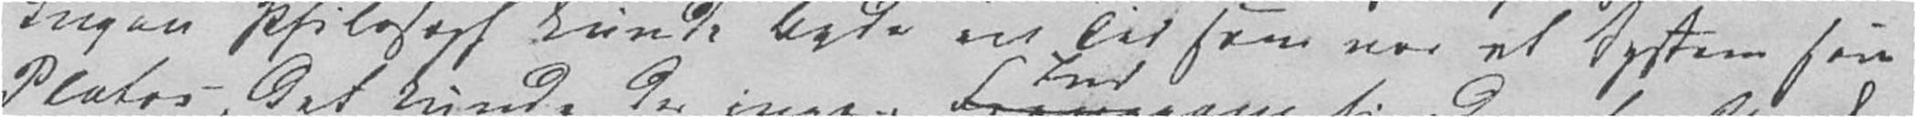Ingen Philosoph kunde byde en Tid som vor et System som
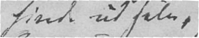finde ud selv,
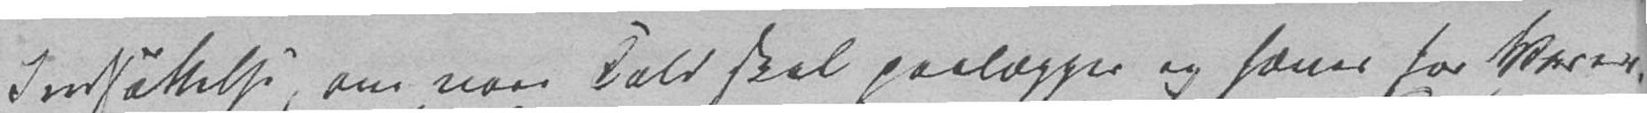Indsættelse, om naar Told skal paalægges og haves for Varer,
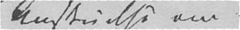Anskuelse om
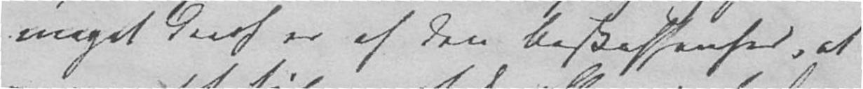meget deraf er af den Beskaffenhed, at
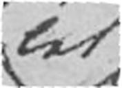let
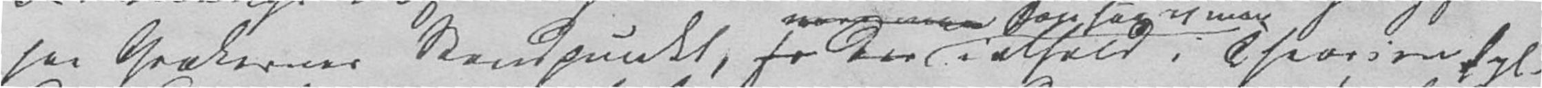paa Grækernes Standpunkt, der ialfald i Theorien hyl-
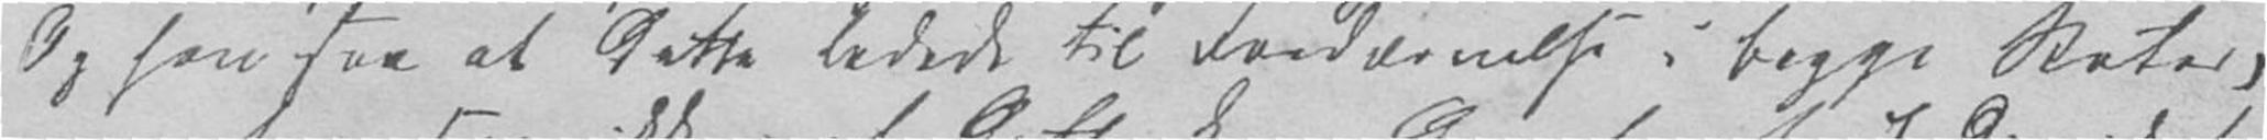Og han saa at dette ledede til Fordærvelse i begge Stater,
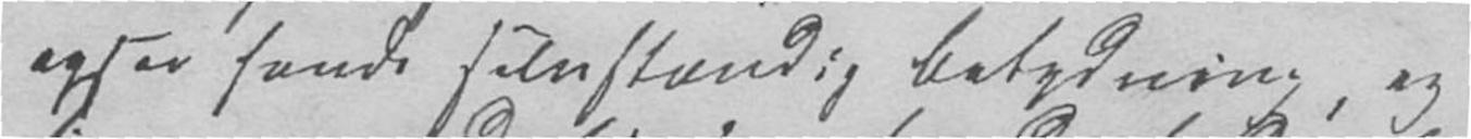ogsaa havde selvstændig Betydning, og
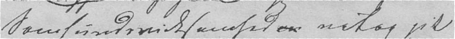Samfundsvirksomheden netop gik
